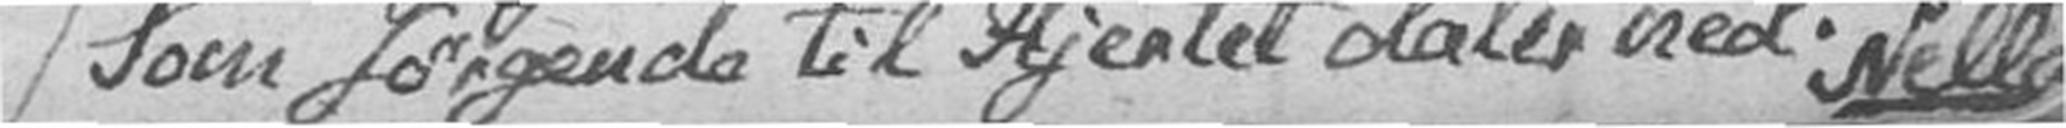Som sørgende til Hjertet daler ned. Nella
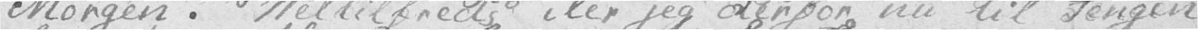Morgen. Veltilfreds iler jeg derfor nu til Sengen
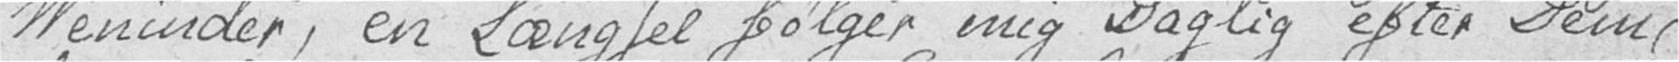Veninder, en Længsel følger mig Daglig efter Dem,
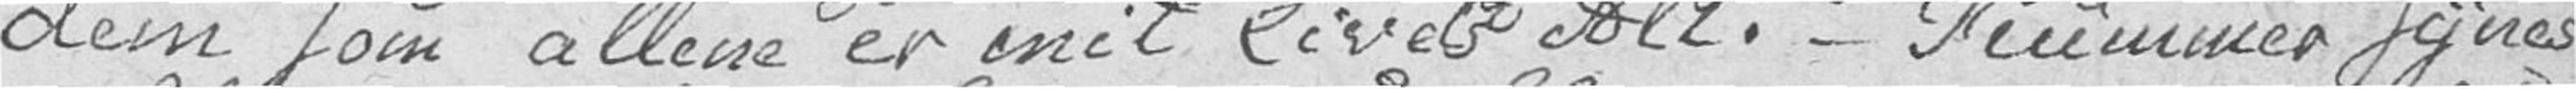dem som allene er mit Livets Alt. – Kummer synes
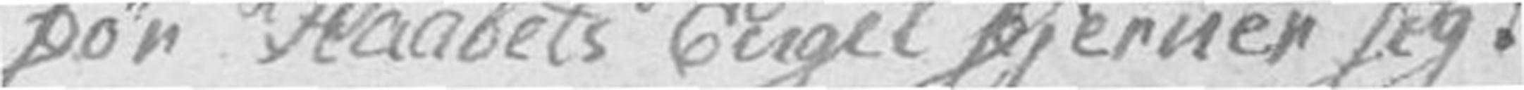før Haabets Engel fjerner sig.
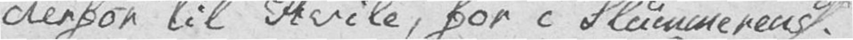derfor til Hvile, for i Slummerens
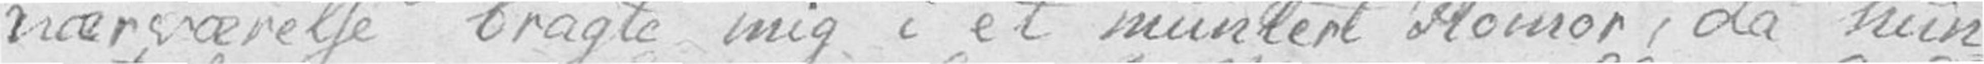nærværelse bragte mig i et muntert Homør, da hun
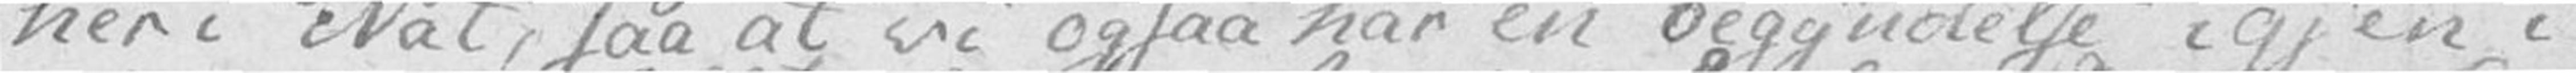her i Nat, saa at vi ogsaa har en begyndelse igjen i
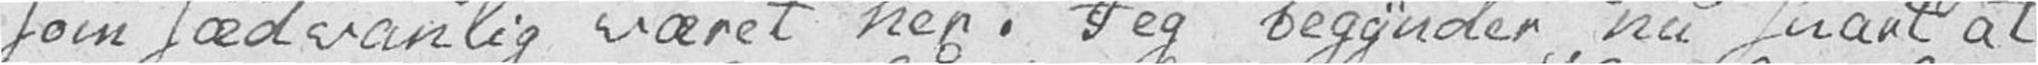som sædvanlig været her. Jeg begynder nu snart at
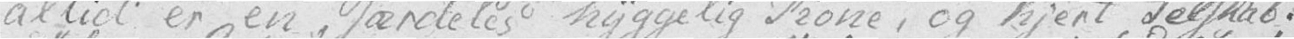altid er en særdeles hyggelig Kone, og Kjert Selskab.
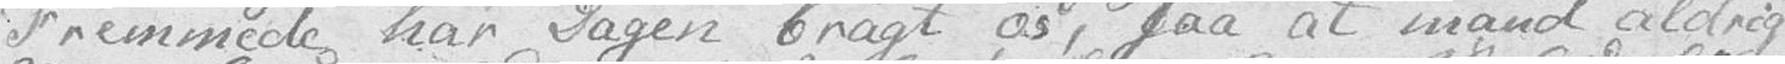Fremmede har Dagen bragt os, saa at mand aldrig
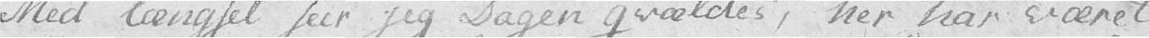Med længsel seer jeg Dagen qvældes, her har været
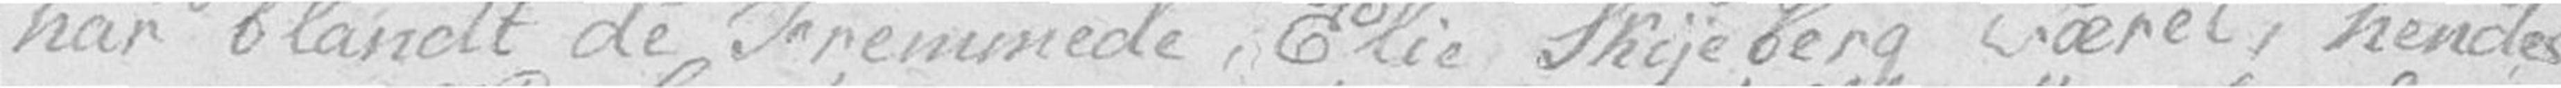har blandt de Fremmede, Elie Skyeberg været, hendes
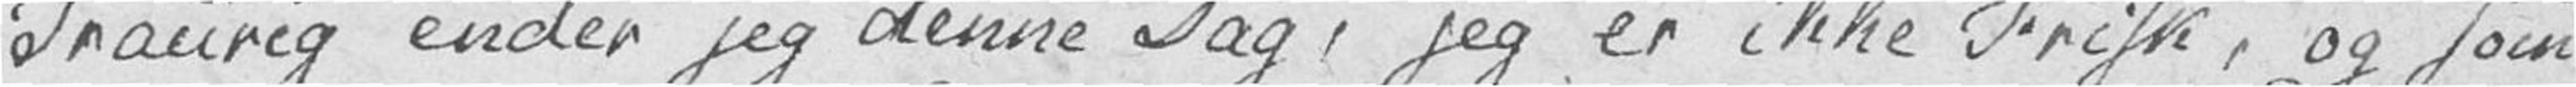Traurig ender jeg denne Dag, jeg er ikke Frisk, og som
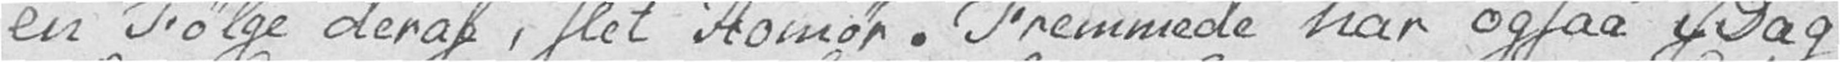en Følge deraf, slet Homør. Fremmede har ogsaa i Dag
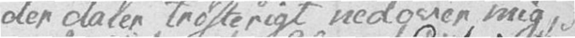der daler trøsterigt nedover mig,
